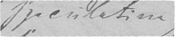speculative
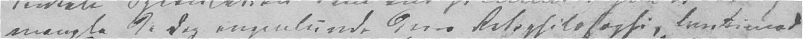mangle de dog ingenlunde deres Retsphilosophi, tvertimod
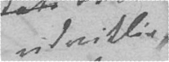udviklin-
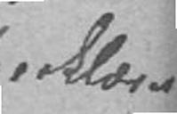erklærer
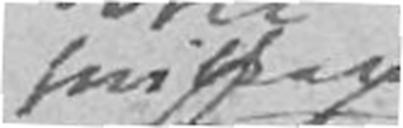hvilken
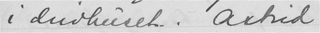i drivhuset. Astrid
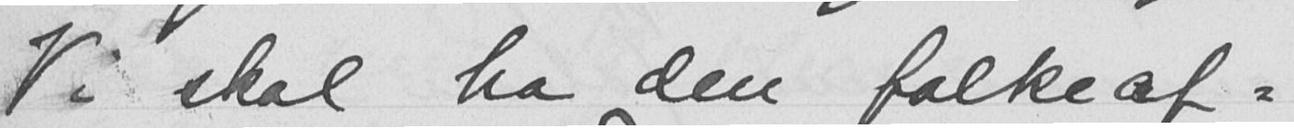Vi skal ha den folkeaf-
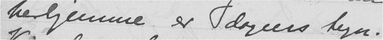herhjemme er dagens tegn.
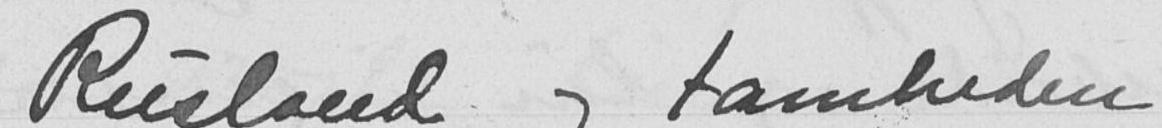Rusland og tamheden
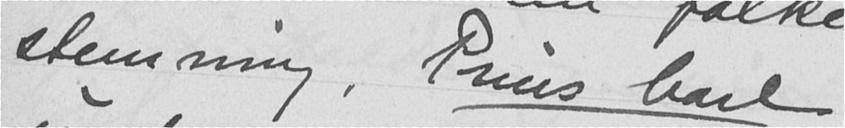stemning, Prins Carl
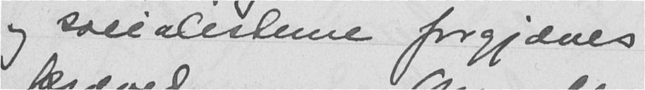og socialisterne forgjæves
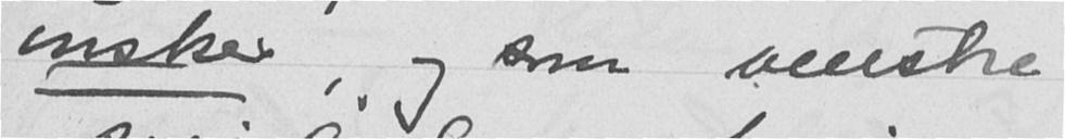ønsker, og som venstre
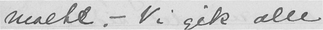malte. - Vi gik alle
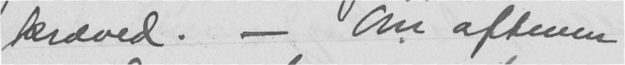kræved. - Om aftenen
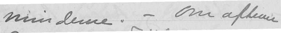minderne. - Om aftenen
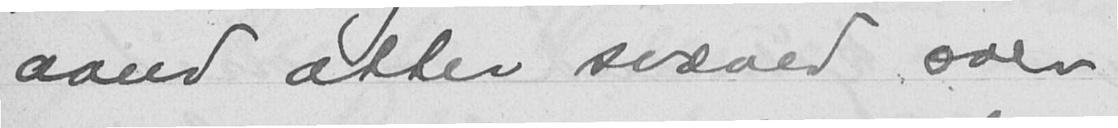aand atter svæved over
